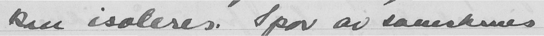kan isoleres. Spor av svenskenes
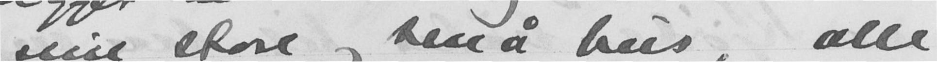sine store og små hus, alle
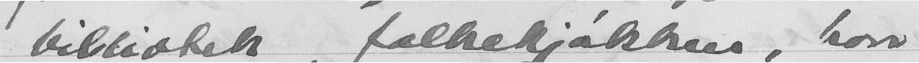bibliotek, folkekjøkken, hvor
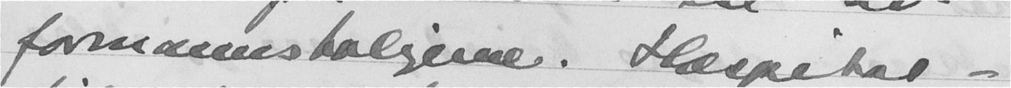formannsboligene. Hospital -
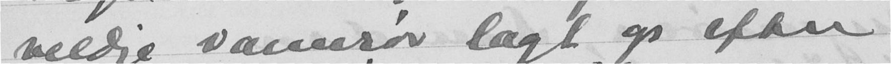veldige vanrør lagt op efter
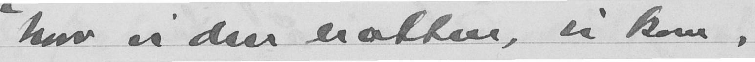hvor vi den natten, vi kom,
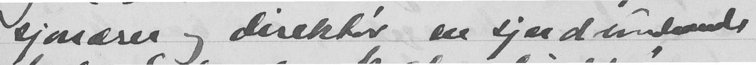sjonæren og direktør en sjeidundrande
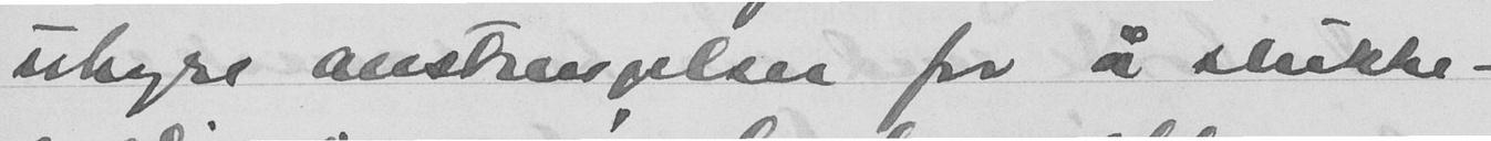uhyre anstrengelser for å slukke -
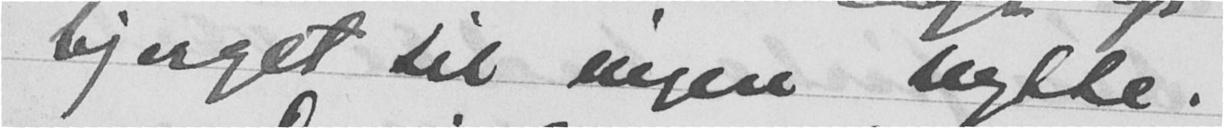bjerget til ingen nytte.
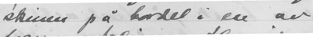skissene på bordet i en av
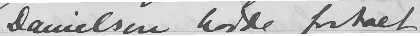Danielsen hadde fortalt
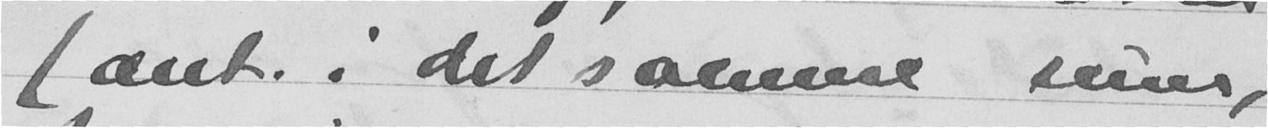(ant. i det samme ruin,
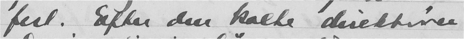fest. Efter den kalte direktøren
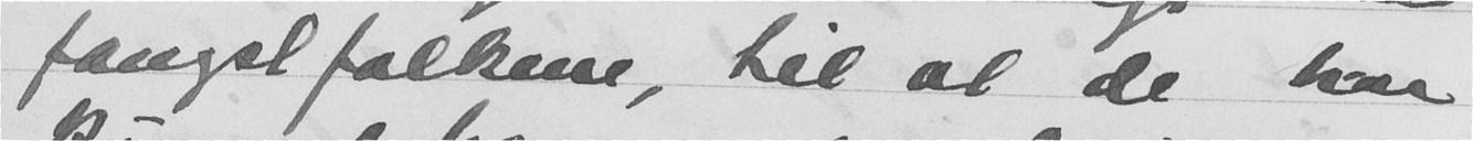fangstfolkene, til at de har
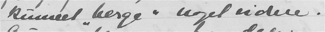kunnet "berge" noget videre.
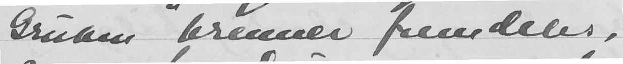Gruben brenner fremdeles,
